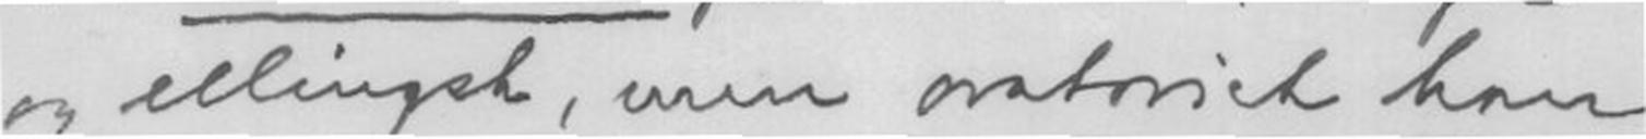og ellingsk, men oratoriet kan
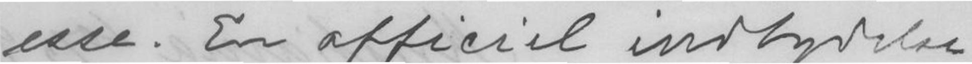esse. En officiel indbydelse
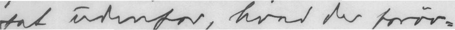sat udenfor, hvad der forøv-
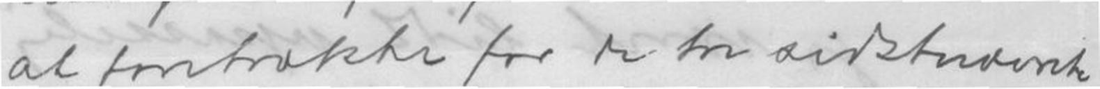at foretrække for de tre sidstnævnte
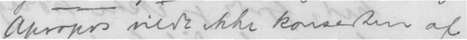Apropos vilde ikke konserten af
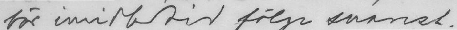bør imidlertid følge snarest.
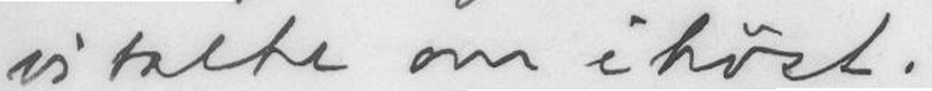vi talte om ihøst.
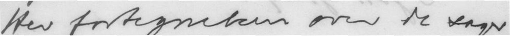Her fortegnelsen over de sager
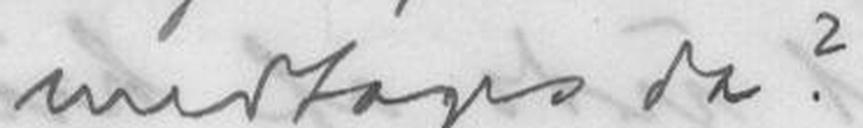undtages da?
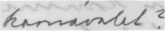karnevalet?
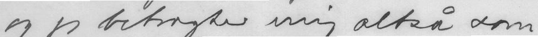og jeg betragter mig altså som
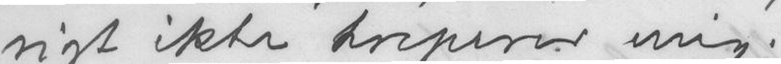rigt ikke kreperer mig.
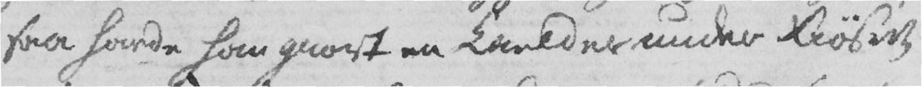saa havde han giort en Kielder under Fiøset
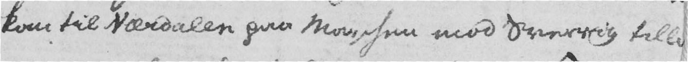kom til Værdalen paa Marchen mod Sverrig tilli-
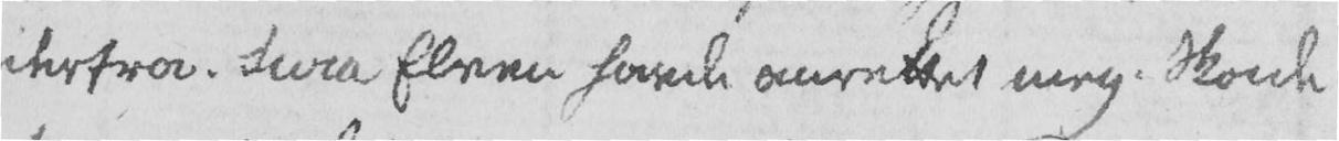derfra. Sura Elven havde anrettet meg. Skade
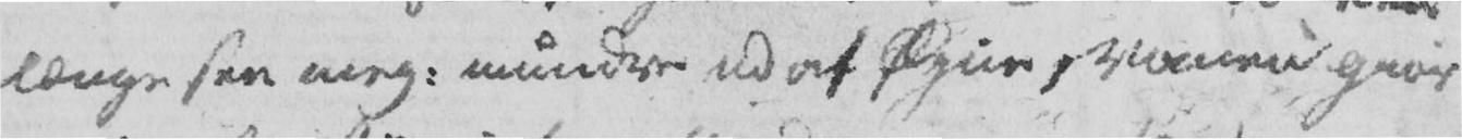længe see meg. mindre ud af Øjne, Vanen giør
